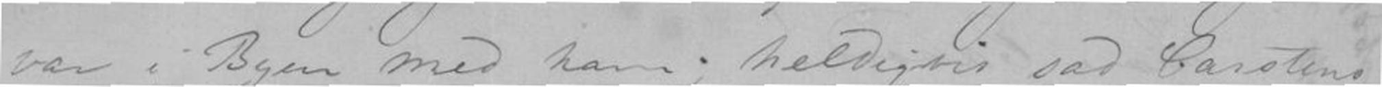var i Byen med ham; heldigvis sad Carstens
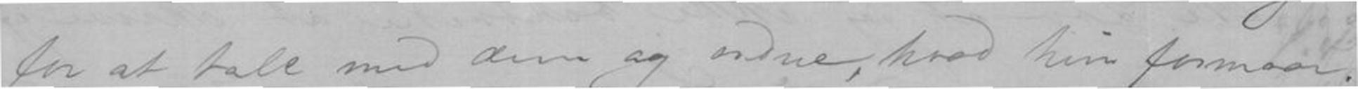for at tale med dem og ordne, hvad hun formaar.
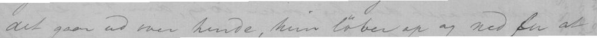det gaar ud over hende, hun løber op og ned for at
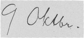9 Oktbr.
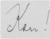Kære!
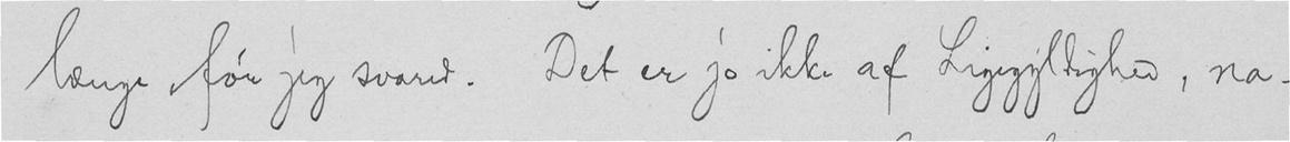længe før jeg svared. Det er jo ikke af Ligegyldighed, na-
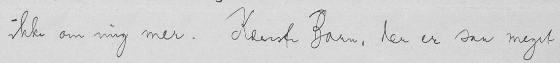ikke om mig mer. Kæreste Barn, der er saa meget
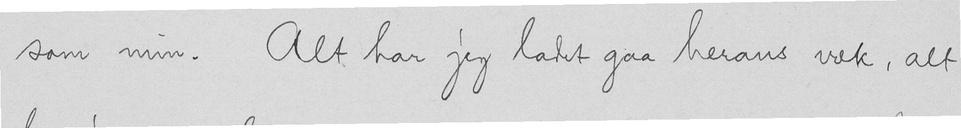som min. Alt har jeg ladet gaa herans væk, alt
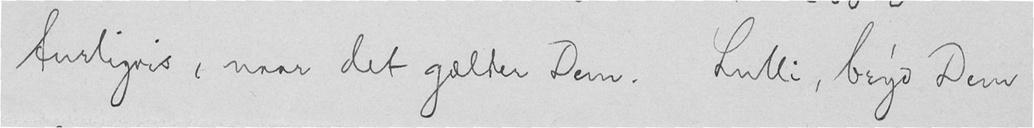turligvis, naar det gælder Dem. Lulli, bryd Dem
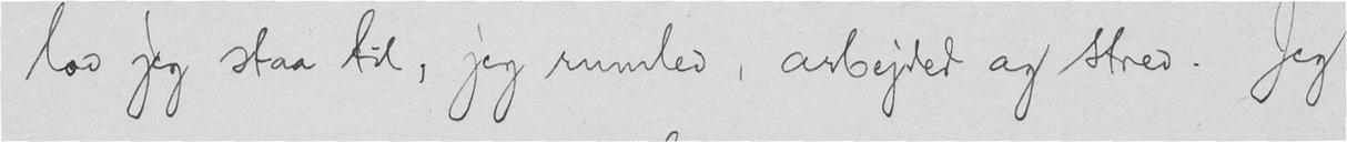lod jeg staa til, jeg rumled, arbejdet og stred. Jeg
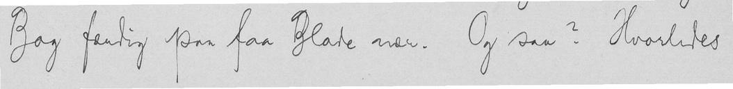Bog færdig paa faa Blade nær. Og saa? Hvorledes
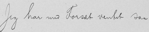Jeg har med Forsæt ventet saa
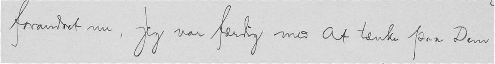forandret nu, jeg var færdig med at tænke paa Dem
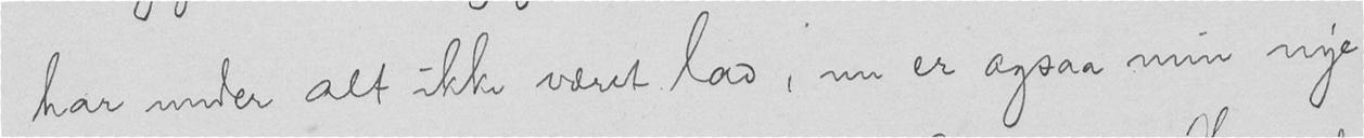har under alt ikke været lad; nu er ogsaa min nye
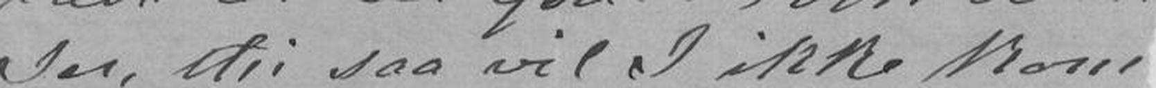Jer, thi saa vil I ikke kom
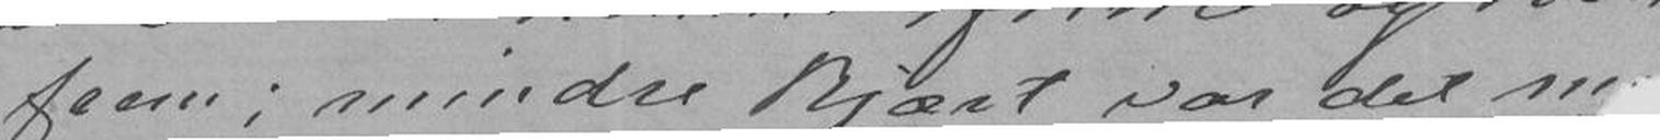frem; mindre kjært var det
